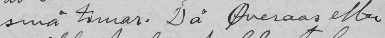små timar. Då Øveraas efter
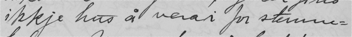ikkje hus å vera i for stemne-
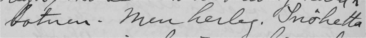botnen. Men herleg. Snöhetta
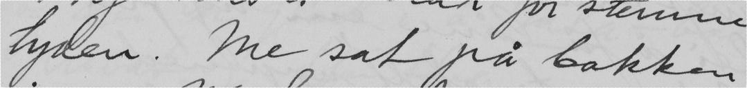tyden. Me sat på bakken
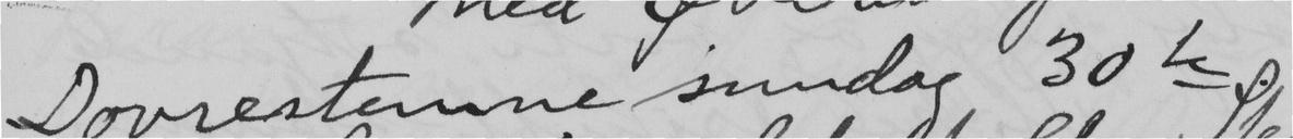Dovrestemne sundag 30 te.
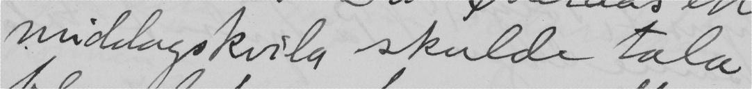middagskvila skulde tala
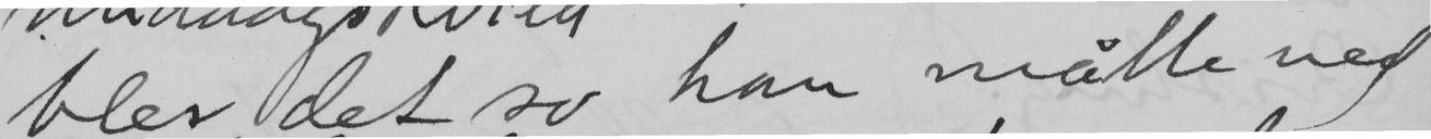blev det so han måtte ned
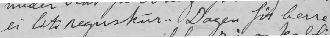ei liti regnskur. Dagen för berre
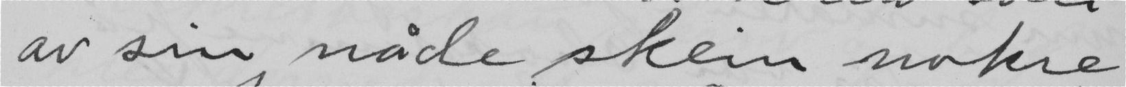av sin nåde skein nokre
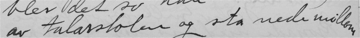av talarstolen og stå nede millom
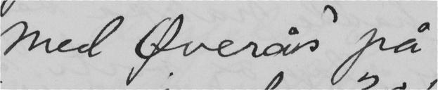Med Øverås på
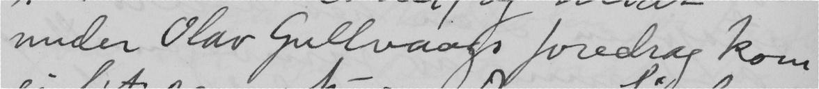under Olav Gullvaags foredrag kom
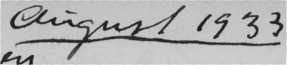August 1933
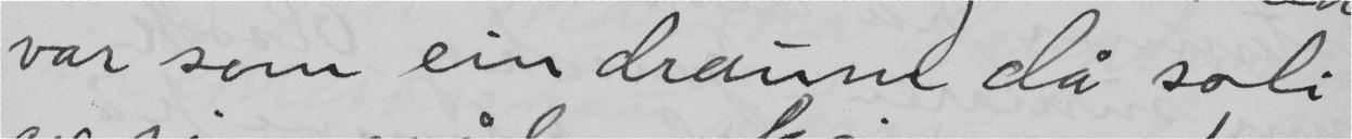var som ein draum då soli
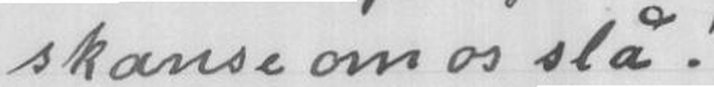skanse om os slå!
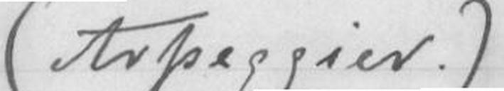(Arpeggier.)
